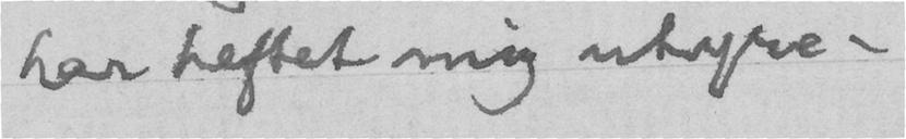har heftet mig uhyre.
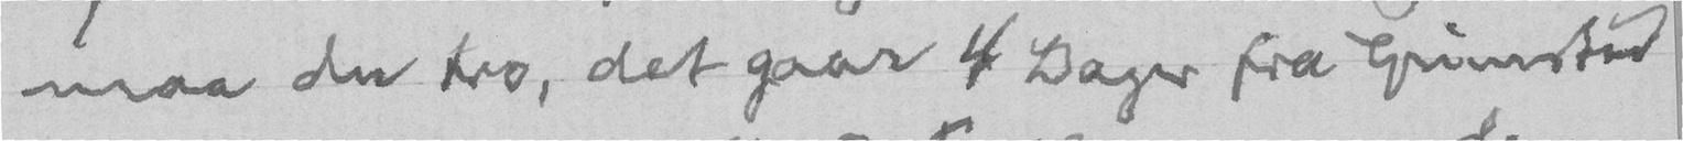maa du tro, det gaar 4 Dager fra Grimstad
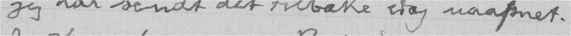jeg har sendt det tilbake idag uaapnet.
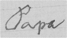Papa
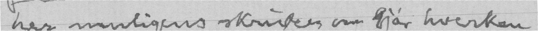her muligens skriver om gjør hverken
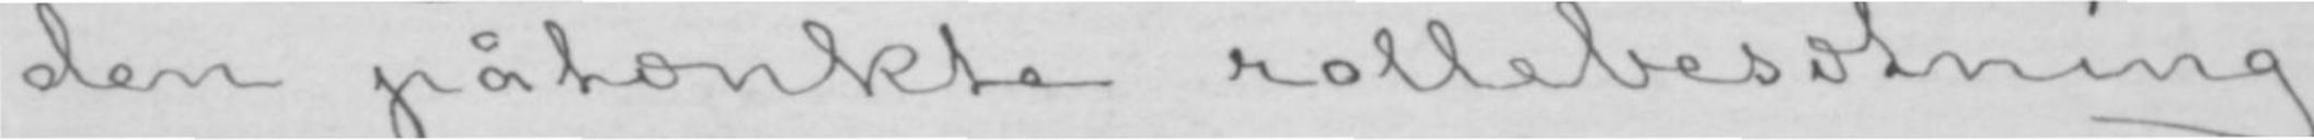den påtænkte rollebesætning,
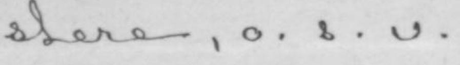stere, o. s. v.
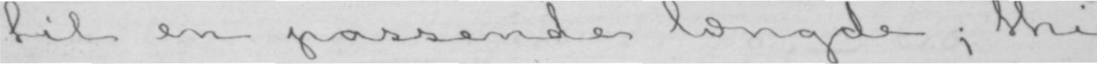til en passende længde; thi
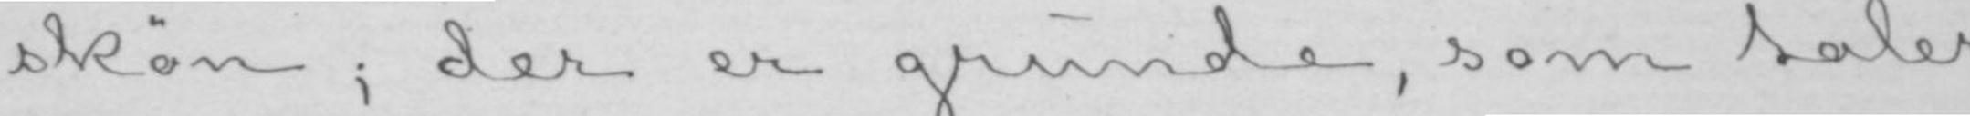skøn; der er grunde, som taler
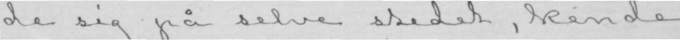de sig på selve stedet, kende
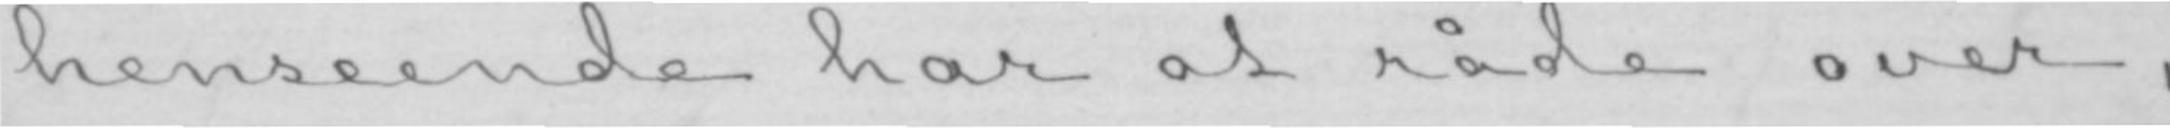henseende har at råde over,
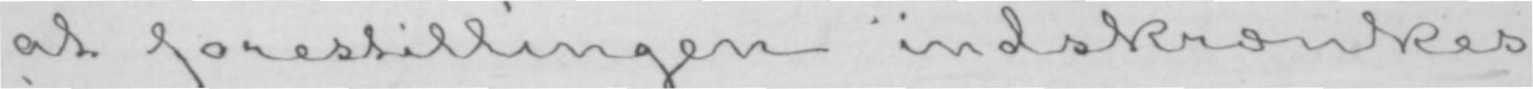at forestillingen indskrænkes
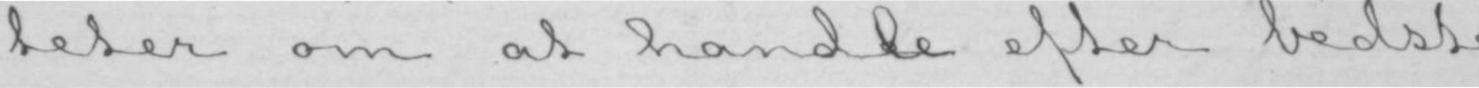teter om at handele efter bedste
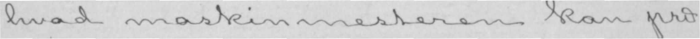hvad maskinmesteren kan præ-
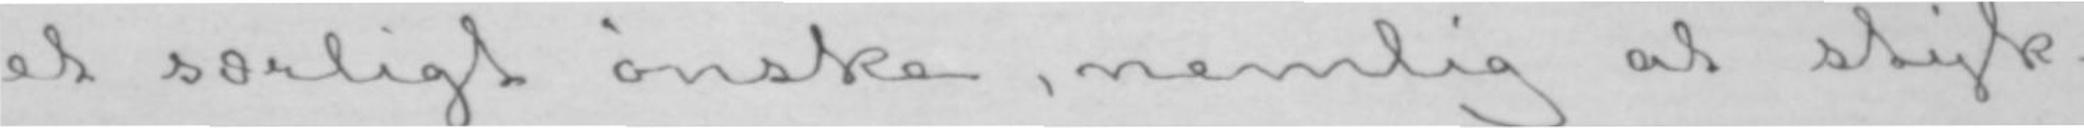et særligt ønske, nemlig at styk-
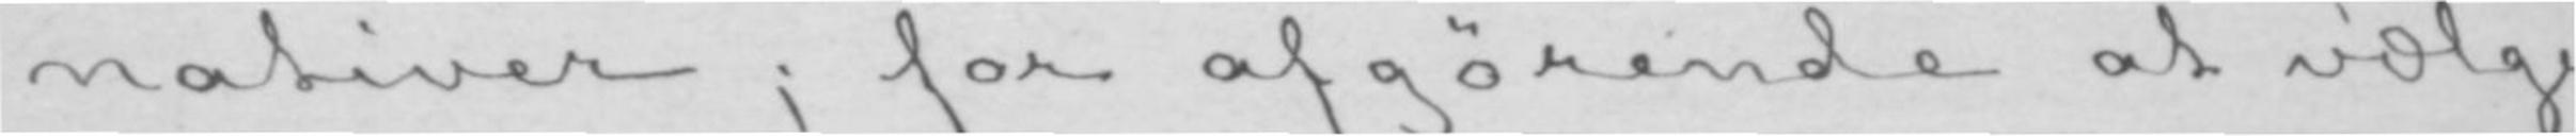nativer; for afgørende at vælge
